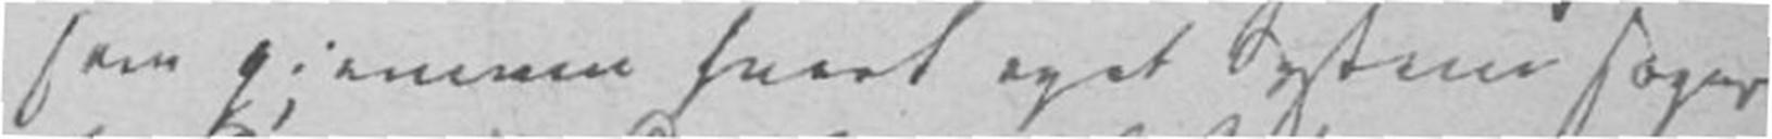som gjennem hvert eget System søger
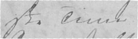ste Time
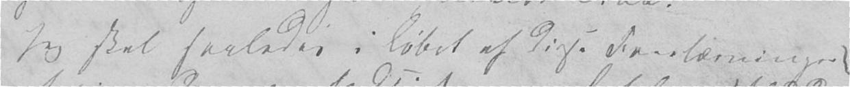Jeg skal saaledes i Løbet af disse Forelæsninger
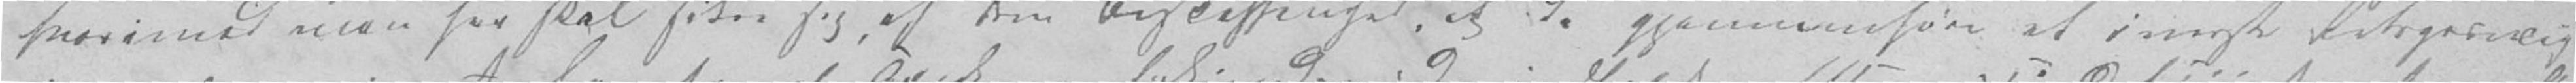hvorimod man her skal sikre sig, af den Beskaffenhed, at de gjennemføre et øverste Retsprincip
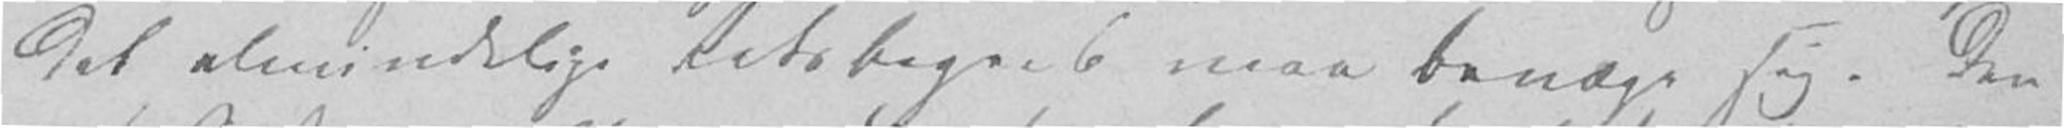det almindelige Retsbegreb maa bevæge sig. Den
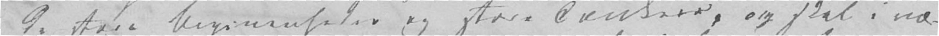de store Begivenheder og store Tænkere, og skal i næ-
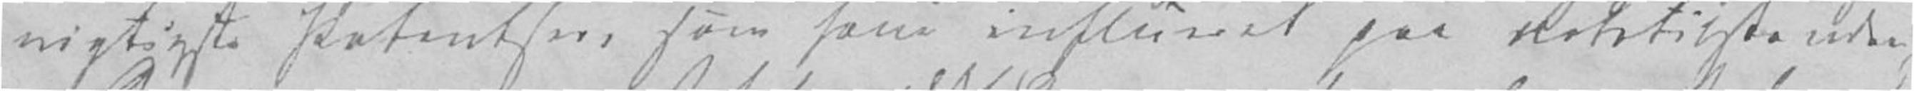vigtigste Potentser, som have influeret paa Retstilstanden
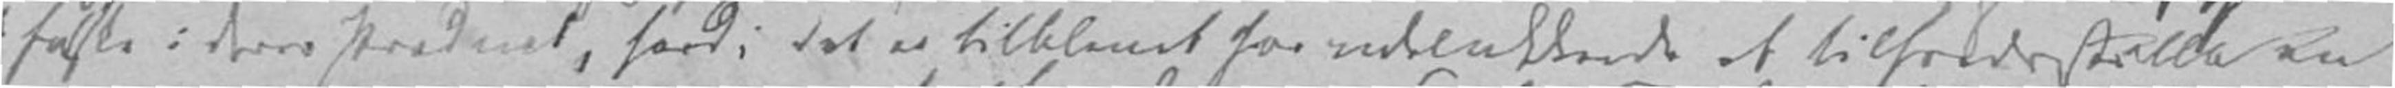falske i deres Product, fordi det er tilblevet for udelukkende at tilfredsstilde en
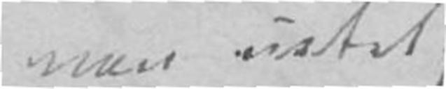man intet
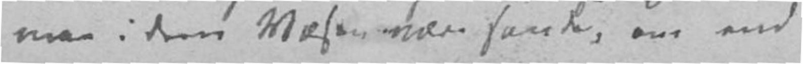maa i dere Væsen være sande, om end
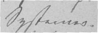Systemer.
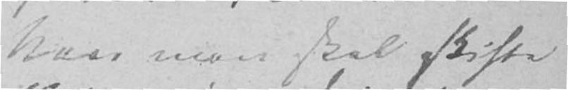Naar man skal skifte
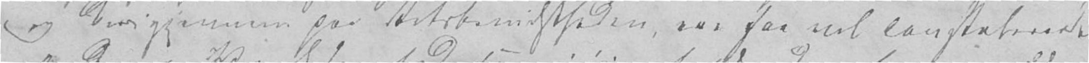og derigjennem paa Retsbevidstheden, ere saa vel constaterede
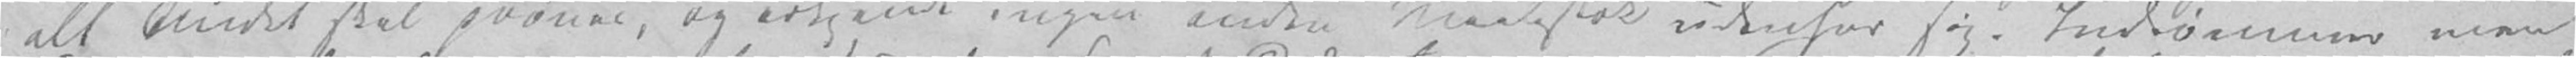alt Andet skal prøves, og erkjende ingen anden Maalestok udenfor sig. Indrømmer man
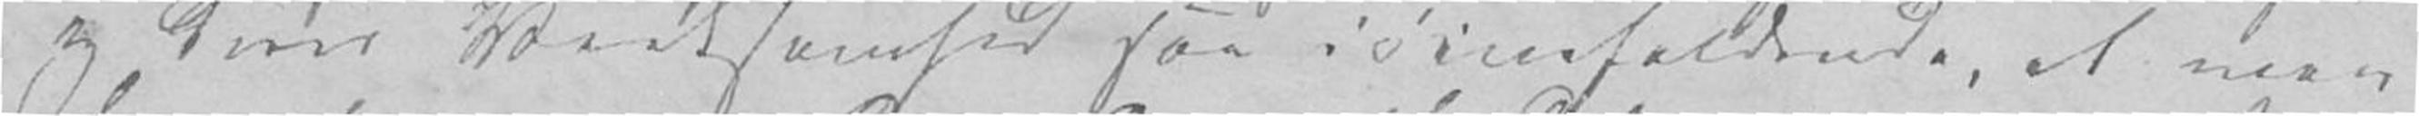og deres Virksomhed saa iøinefaldende, at man
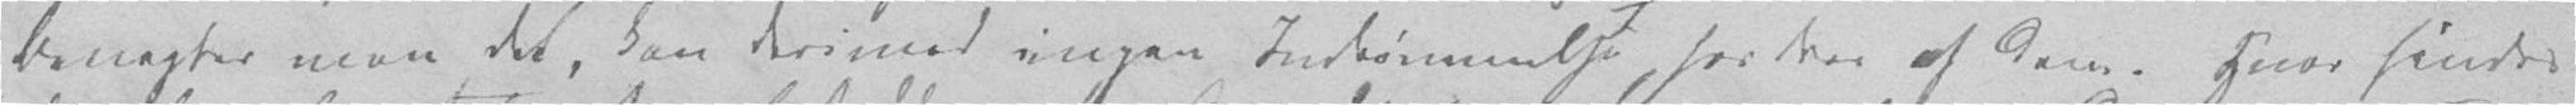Benægter man det, kan derimod ingen Indrømmelse fordres af dem. Hvor findes
